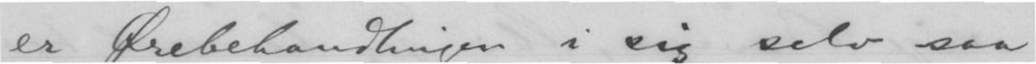er Ørebehandlingen i sig selv saa
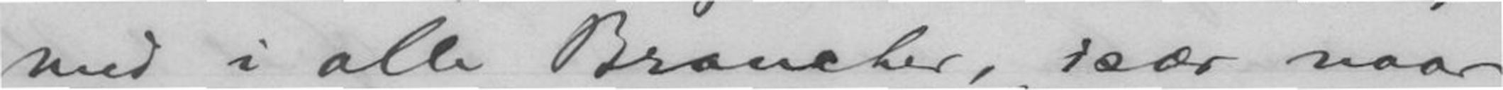med i alle Brancher, især naar
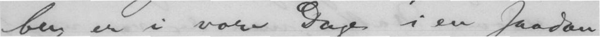ben er i vore Dage i en saadan
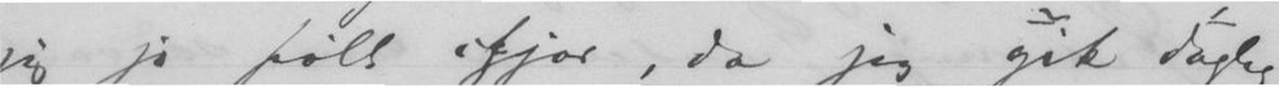jeg jo følt ifjor, da jeg gik daglig
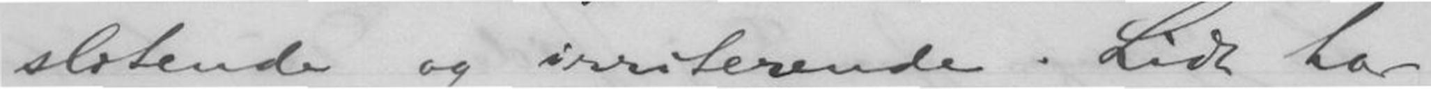slitende og irriterende. Lidt har
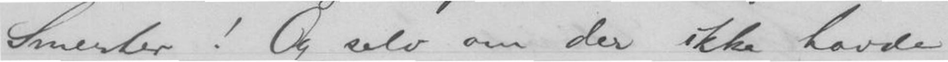Smerter! Og selv om der ikke havde
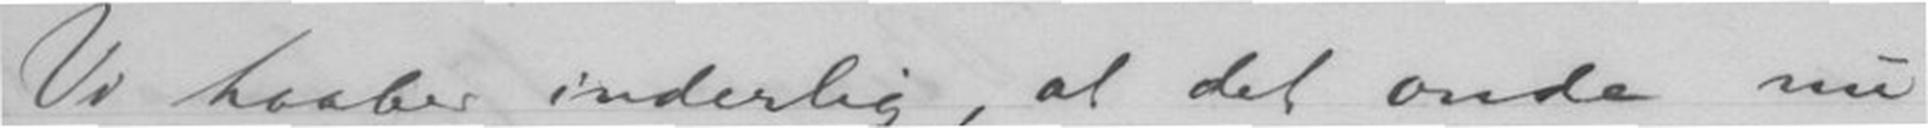Vi haaber inderlig, at det onde nu
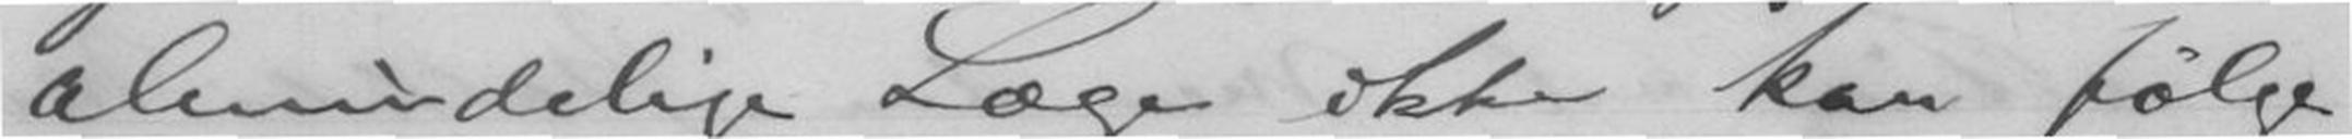almindelige Læge ikke kan følge
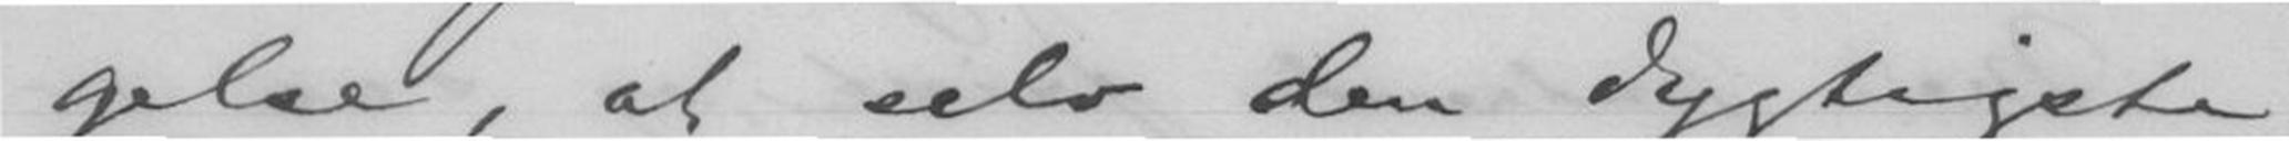gelse, at selv den dygtigste
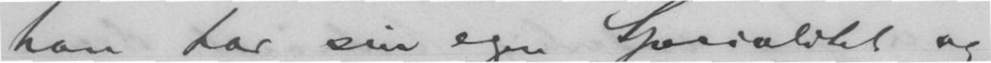han tar sin egen Specialitet og
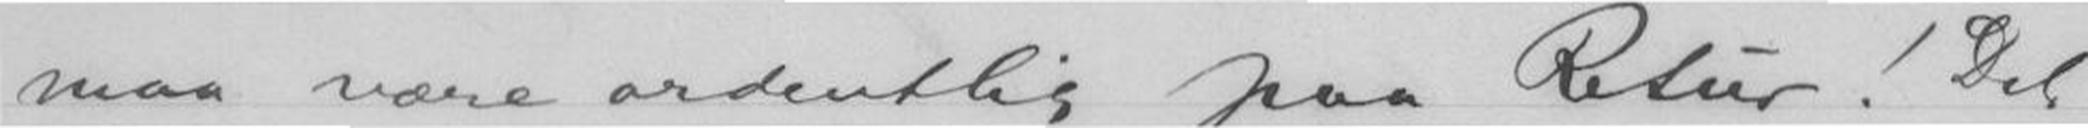maa være ordenlig paa Retur! Det
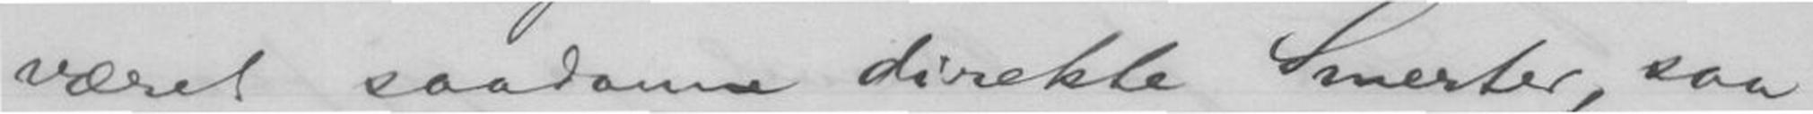været saadanne direkte Smerter, saa
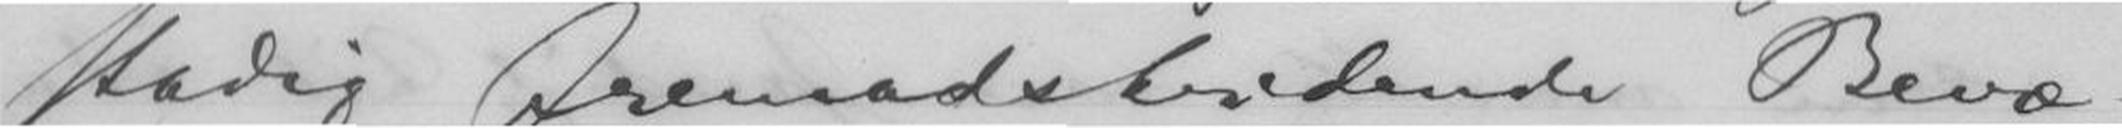stadig fremadskridende Bevæ-
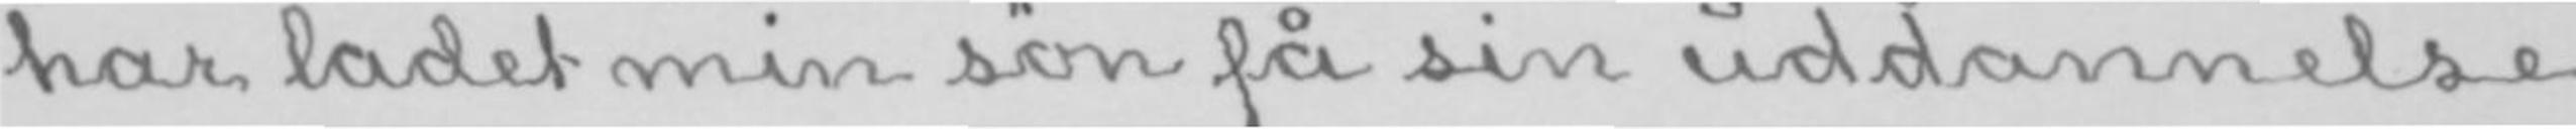har ladet min søn få sin uddannelse
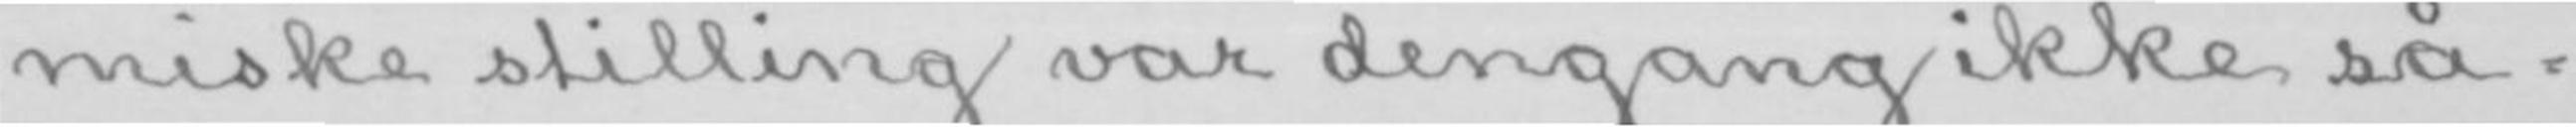miske stilling var dengang ikke så-
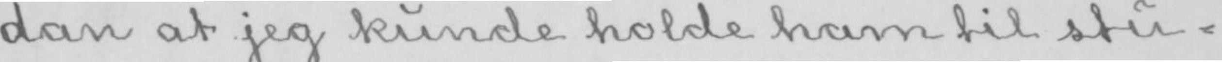dan at jeg kunde holde ham til stu-
